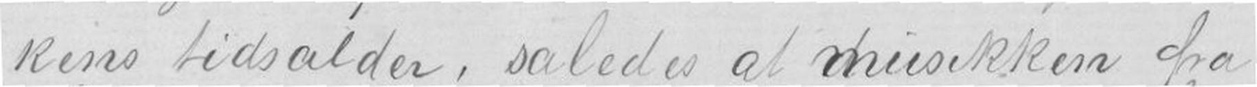kens tidsalder, således at musikken fra
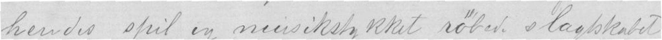hendes spil og musikstykket røbede slægtskabet
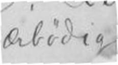Ærbødig
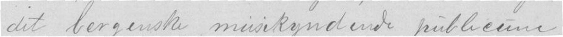dit bergenske musikyndende publicum
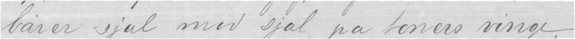bärer sjæl mod sjæl på toners vinge.
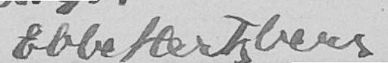Ebbe Hertzberg.
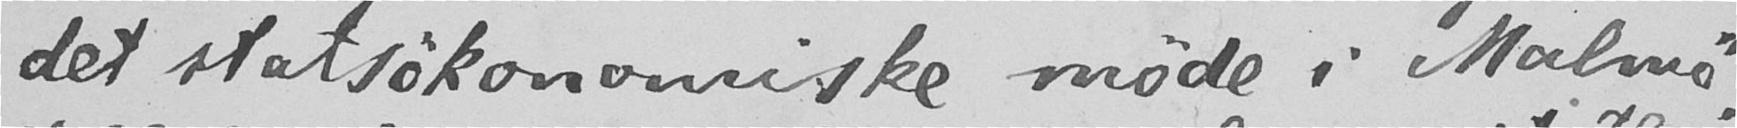det statsøkonomiske møde i Malmö,
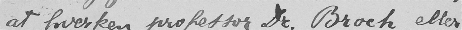at hverken professor Dr. Broch eller
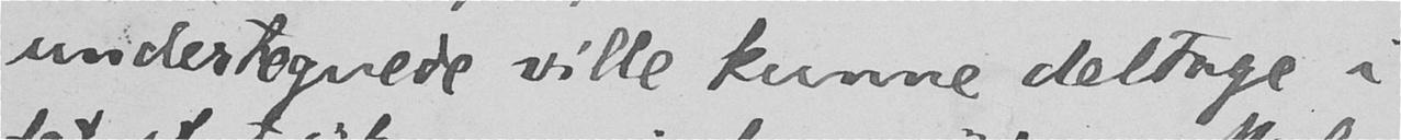undertegnede ville kunne deltage i
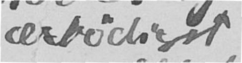ærbødigst
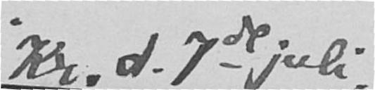Kr. d. 7de juli.
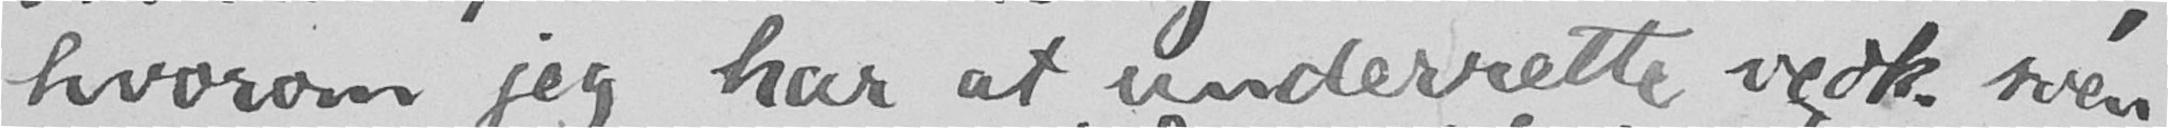hvorom jeg har at underrette vedk. sven-
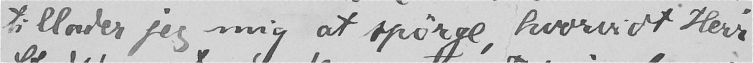tillader jeg mig at spørge, hvorvidt Herr
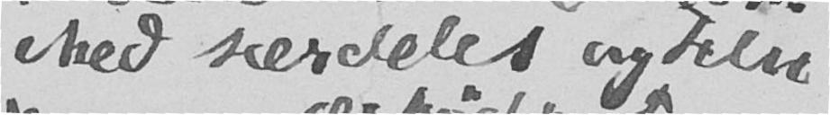Med særdeles agtelse
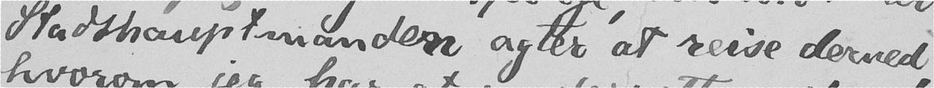Stadshauptmanden agter at reise derved,
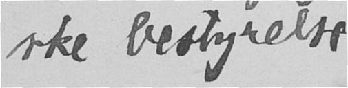ske bestyrelse.
